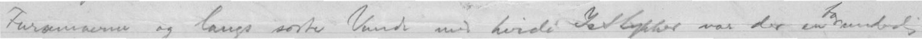Furumoerne og langs sorte Vand med hvide Isstykker var der en forunderlig
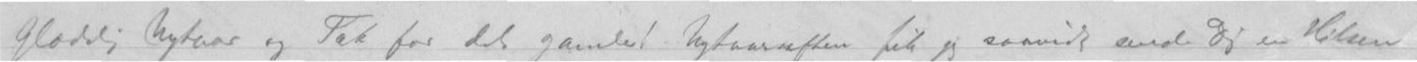Glædelig Nytaar og Tak for det gamle! Nytaarsaften fik jeg saavidt sendt Dig en Hilsen
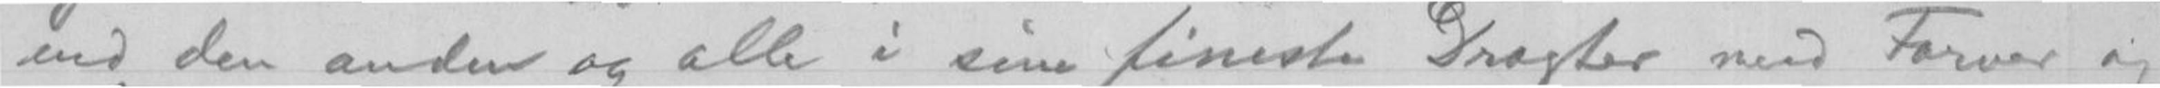end den anden og alle i sine fineste Dragter med Farver og
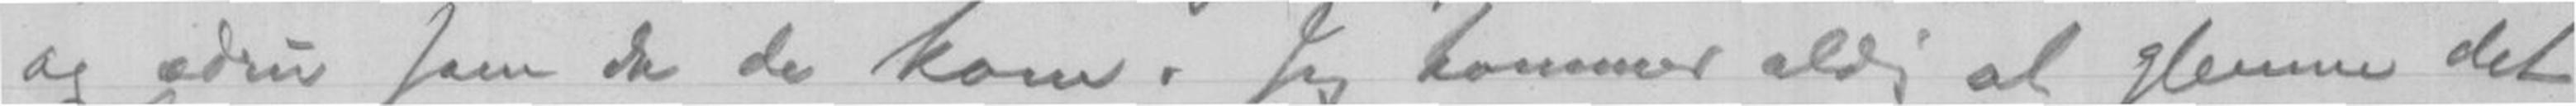og ædru som da de kom. Jeg kommer aldrig at glemme det
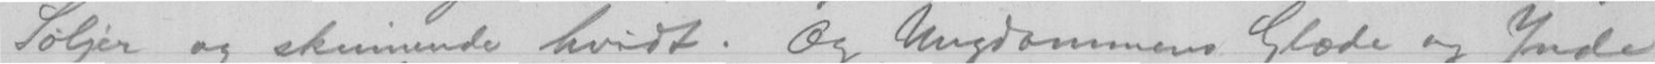Søljer og skinnende hvidt. Og Ungdommens Glæde og Ynde
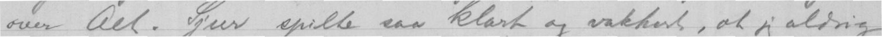over Alt. Sjur spilte saa klart og vakkert, at jeg aldrig
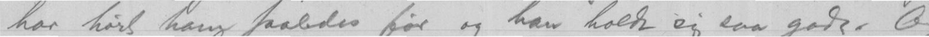har hørt ham saaledes før og han holdt sig saa godt. Og
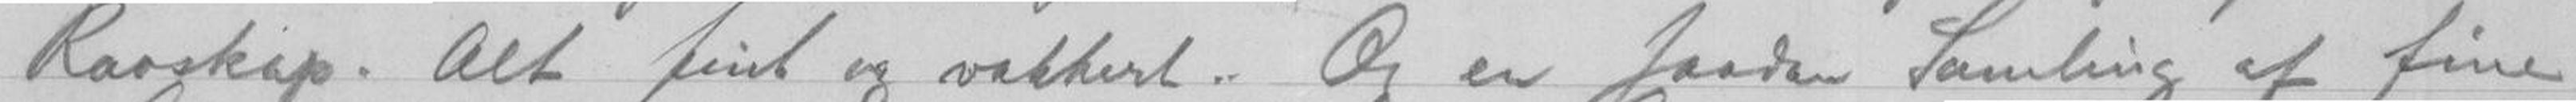Raaskap. Alt fint og vakkert. Og en saadan Samling af fine
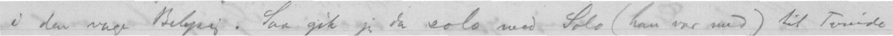i den vage Belysning. Saa gik jeg da solo med Solo (han var med) til Tvinde,
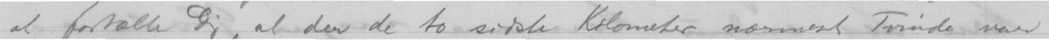at fortælle Dig, at der de to sidste Kilometer nærmest Tvinde var
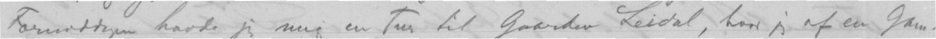Formiddagen havde jeg mig en Tur til Gaarden Leidal, hvor jeg af en Gam-
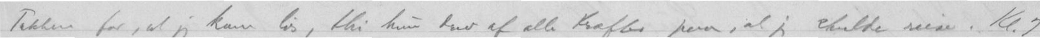Takken for, at jeg kom løs, thi hun drev af alle Kræfter paa, at jeg skulde reise. Kl. 7
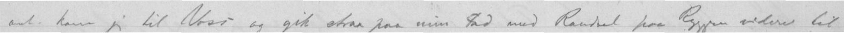omt. kom jeg til Voss og gik strax paa min Fod med Randsel paa Ryggen videre til
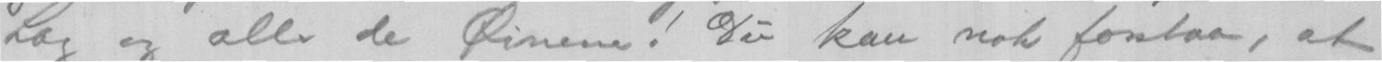Lag og alle de Øinene! Du kan nok forstaa, at
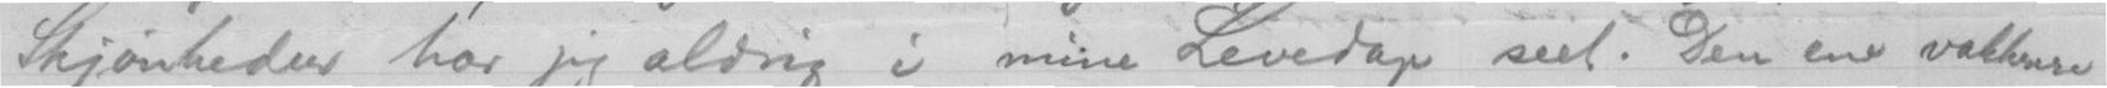Skjønheder har jeg aldrig i mine Levedage seet. Den ene vakkrere
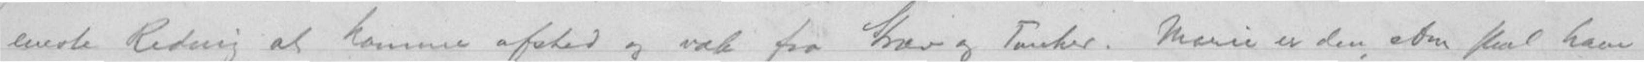eneste Redning at komme afsted og væk fra Strev og Tanker. Marie er den, som skal have
